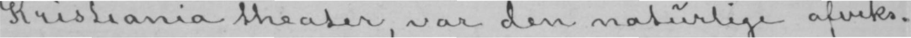Kristiania theater, var den naturlige afveks-
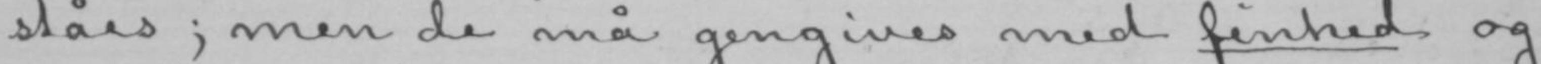ståes; men de må gengives med finhed og
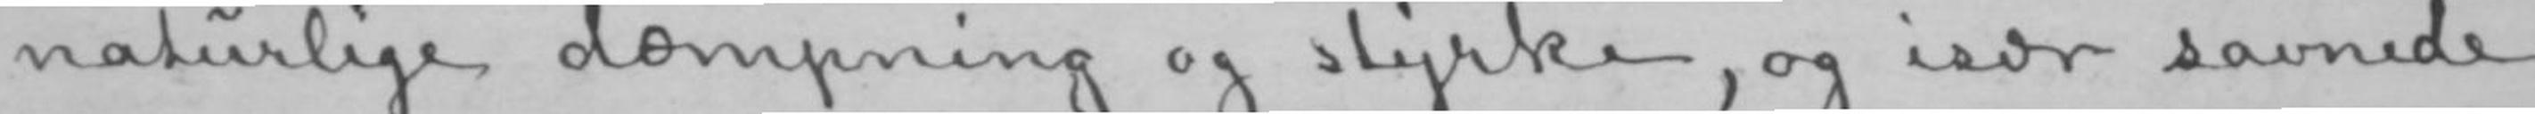naturlige dæmpning og styrke, og især savnede
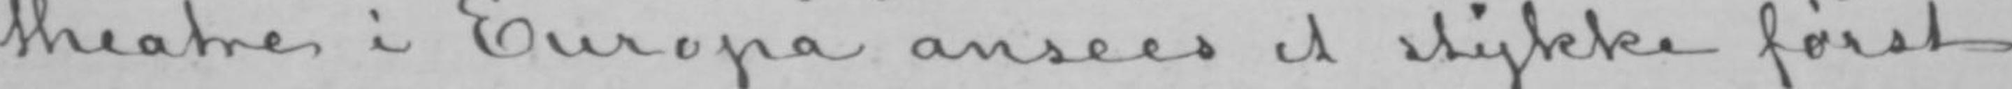theatre i Europa ansees et stykke først
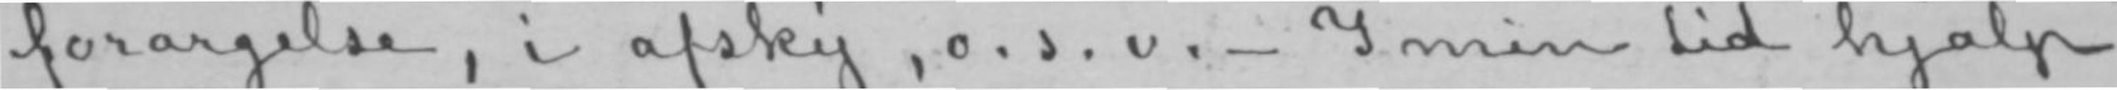forargelse, i afsky, o. s. v.- I min tid hjalp
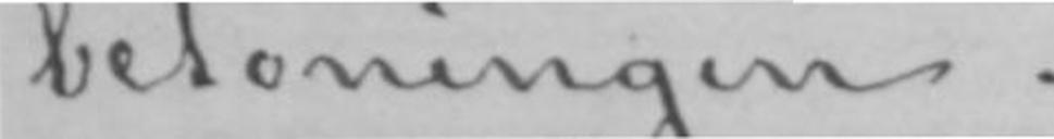betoningen.
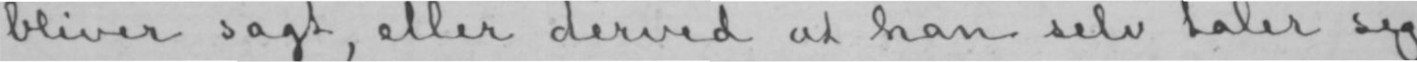bliver sagt, eller derved at han selv taler sig
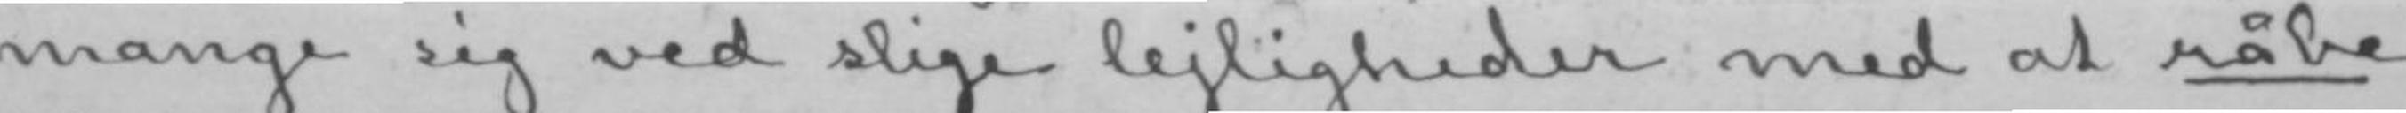mange sig ved slige lejligheder med at råbe
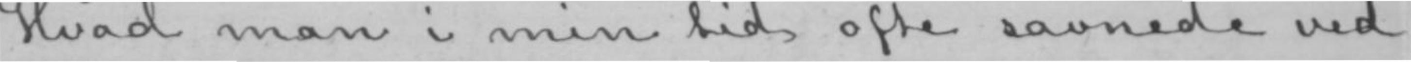Hvad man i min tid ofte savnede ved
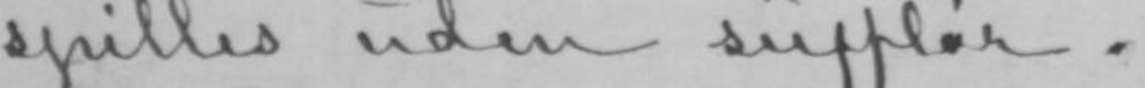spilles uden sufflør.
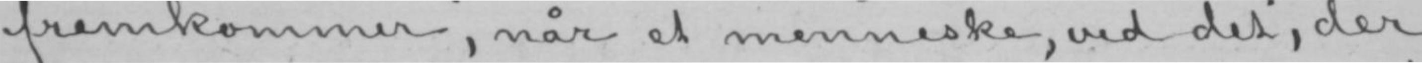fremkommer, når et menneske, ved det, der
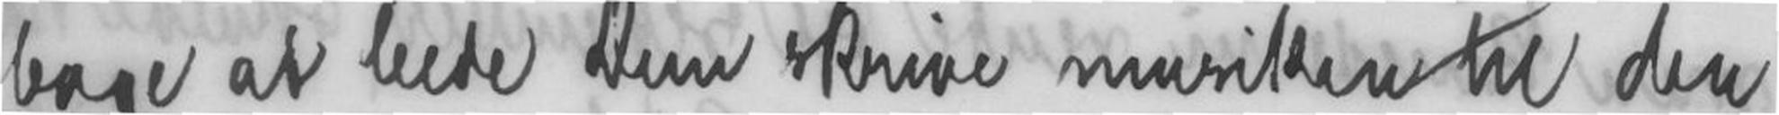bage at bede Dem skrive musiken til den
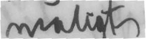muligt.
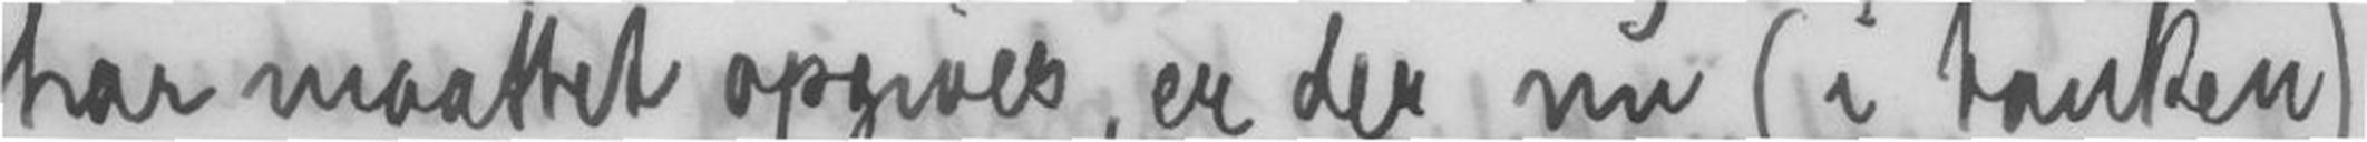har maattet opgives, er der nu (i tanken)
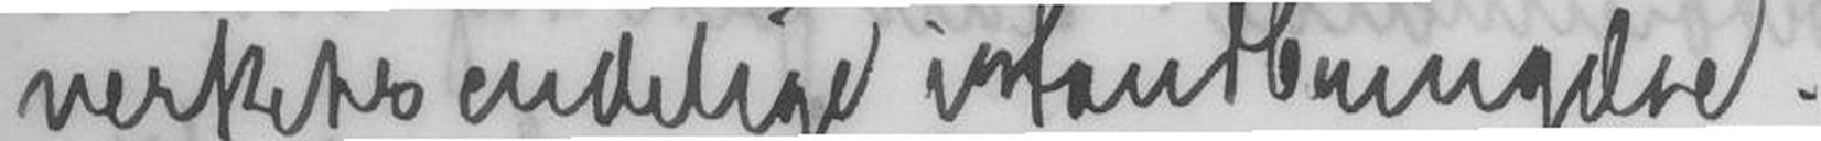verkets endelige istandbringære.
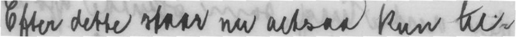Efter dette staar nu altsaa kun til-
